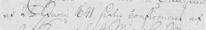af 2den Juni 1641, siden confirmeret af
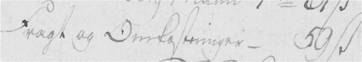Fragt og Omkostninger - 59 s
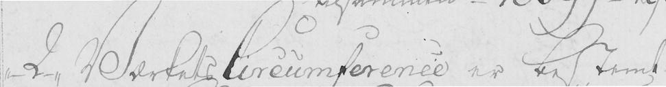"-2-" Værkets Circumference er bestemt
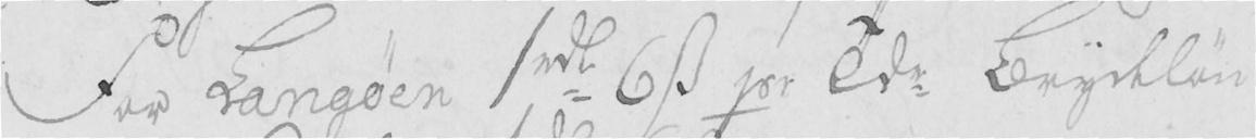For Langøen 1 rdl 6 s pr Tdr Brydeløn
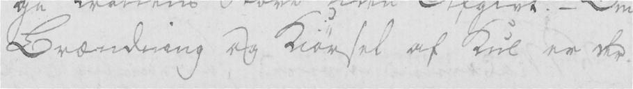Brændning og Kiørsel af Kul er der
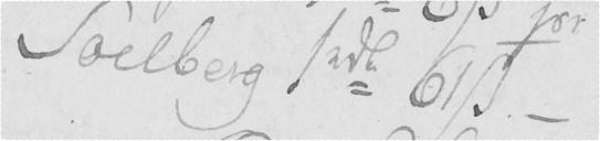Soelberg 1 rdl 61 s. -
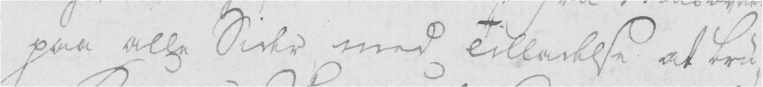paa alle Sider med Tilladelse at bru-
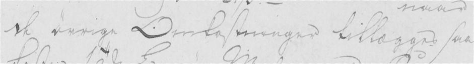de øvrige Omkostninger tillægges saa
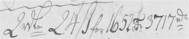2 rde 24 s for 1652 Tdr 3717 rde
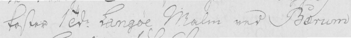koster 1 Tdr Langøe Malm ved Bærum
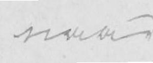naar
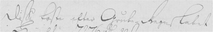Disse koste efter Grube-Regnskabet
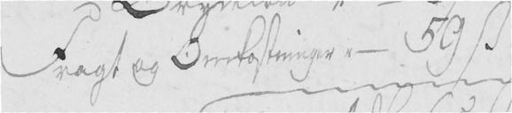Fragt og Omkostninger "- 59 s
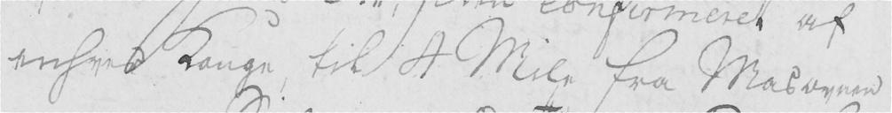enhver Konge, til 4 Mile fra Masovnen
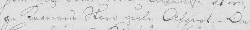ge Kronens Skove uden Afgivt. - Om
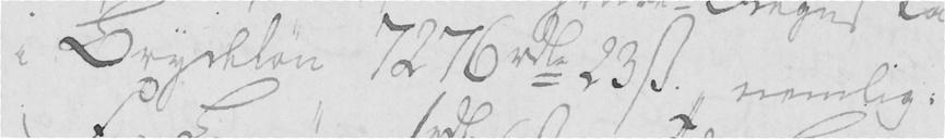i Brydeløn 7276 rde 23 s., nemlig.
